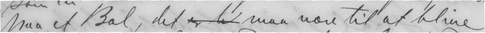paa et Bal, det er le maa være til at blive
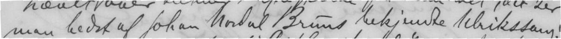man bedst af Johan Nordal Bruns bekjendte Ulrikssang!
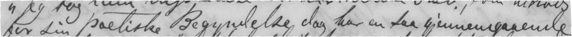for sin poetiske Begyndelse dog har en saa gjennemgaaende
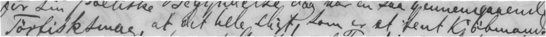Tørfisksmag, at det hele Digt, som er et pent Kjøbmands-
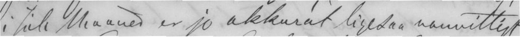i juli Maaned er jo akkurat ligesaa vanvittigt
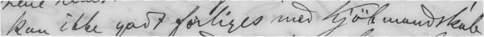kan ikke godt forliges med Kjøbmandskab
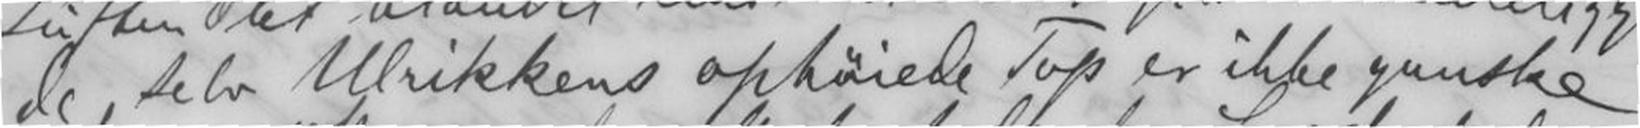de, selv Ulrikkens ophøiede Top er ikke ganske
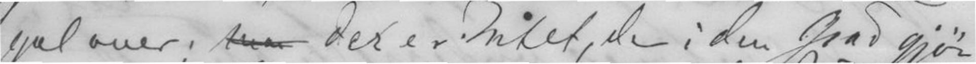gal over; men der er Intet, der i den Grad gjør
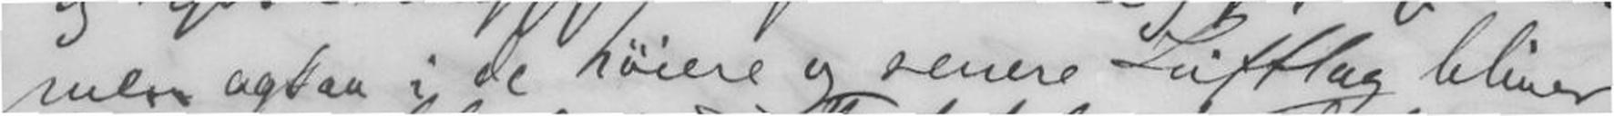men ogsaa i de høiere og senere Luftlag bliver
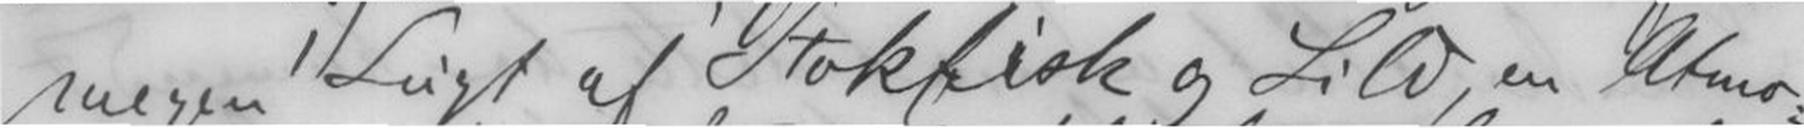megen Luft af Stokfisk og Sild, en Atmo-
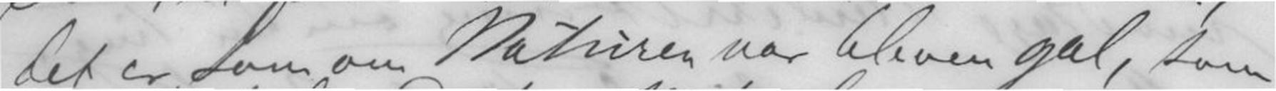der er som Naturen var bleven gal, som
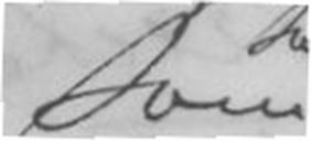som
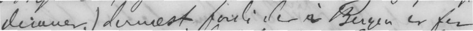derover;) dernest fordi der i Bergen er for
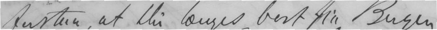forstaa, at Du længes bort fra Bergen,
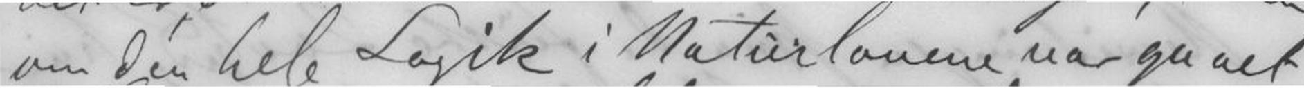om den hele Logik i Naturlovene var gaaet
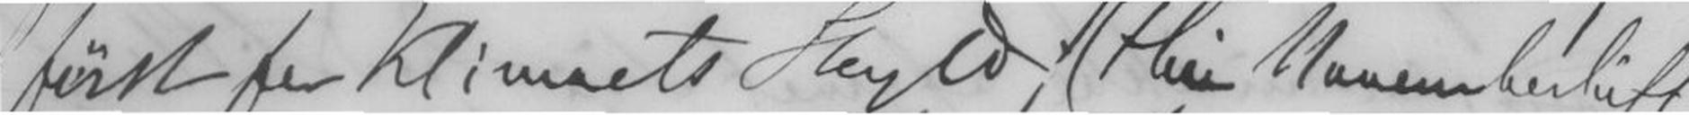først for Klimaets Skyld; (thi Novemberluft
